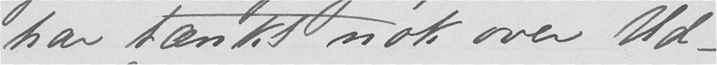har tænkt nok over Ud-
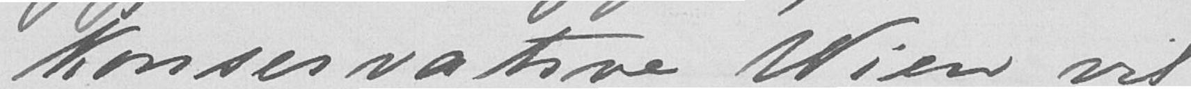konservative Wien vil
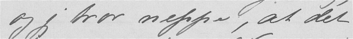og jeg tror neppe, at det
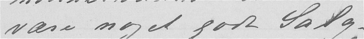være noget godt Salg-
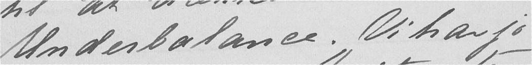Underbalance. Vi har jo
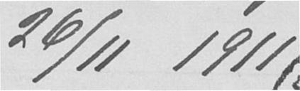26/11 1911
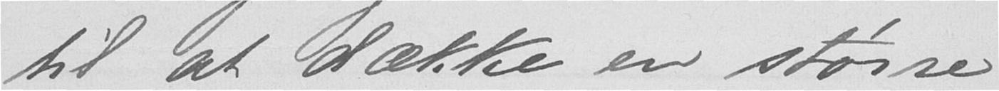til at dække en større
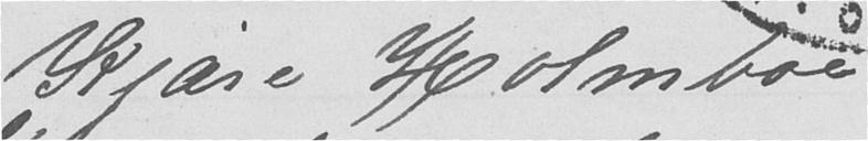Kjære Holmboe
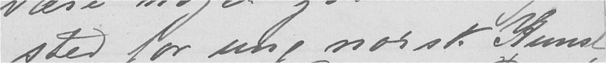sted for ung norsk Kunst,
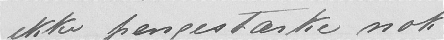ikke pengestærke nok
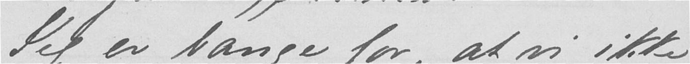Jeg er bange for, at vi ikke
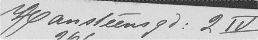Hansteensgd. 2 IV
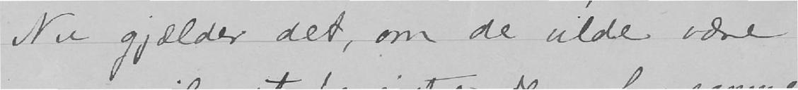Nu gjælder det, om de vilde være
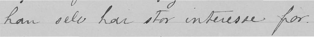han selv har stor interesse for.
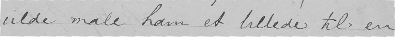vilde male ham et billede til en
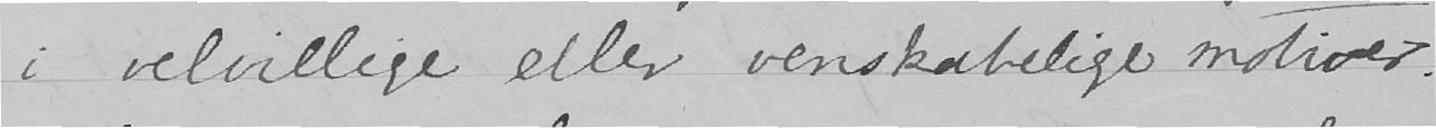i velvillige eller venskabelige motiver.
